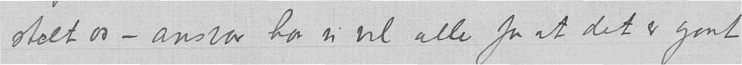stelt os – ansvar har vi vel alle for at det er gaat
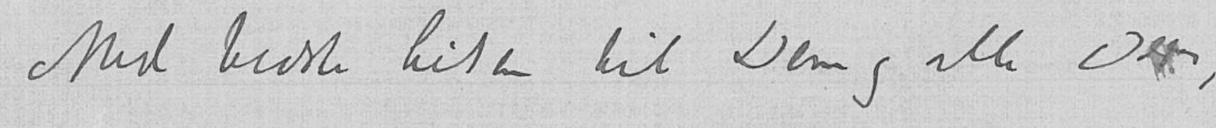Med bedste hilsen til Dem og alle Deres,
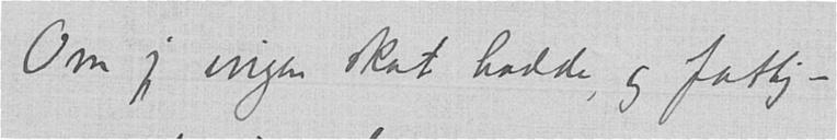Om jeg ingen skat hadde, og fattig-
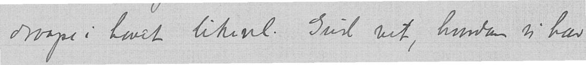draape i havet likevel. Gud vet hvordan vi har
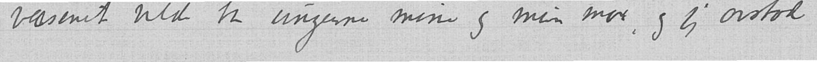væsenet vilde ha ungerne mine og min mor, og jeg avstod
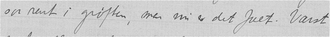saa rent i grøften, men nu er det fælt. Værst
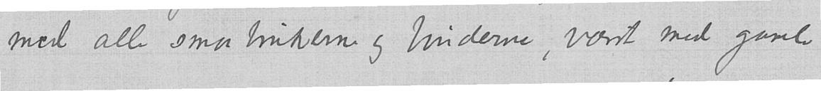med alle smaabrukerne og bønderne, værst med gamle
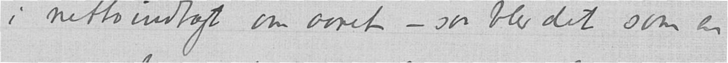i nettoindtægt om aaret – saa blev det som en
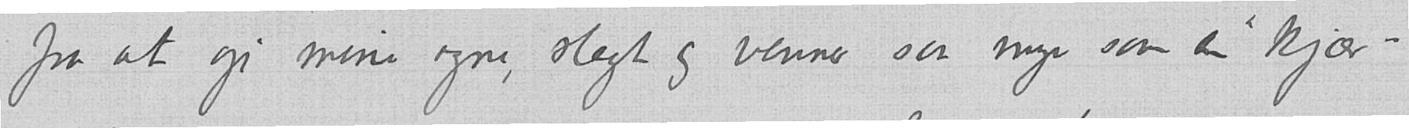fra at gi mine egne, slegt og venner saa mye som en "kjær-
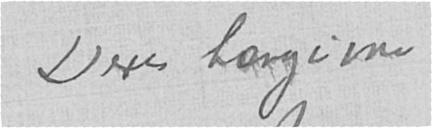Deres hængivne
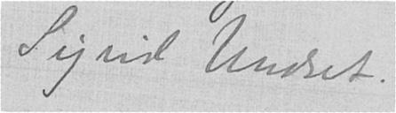Sigrid Undset.
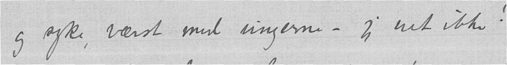og syke, værst med ungerne – jeg vet ikke!
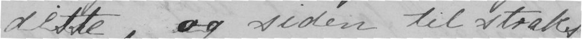dette, og siden til straks
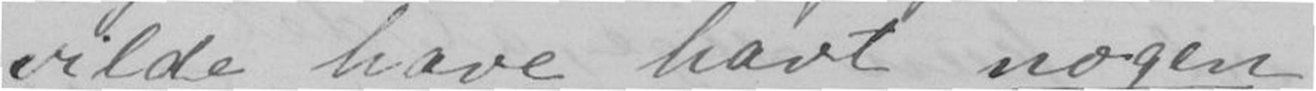vilde have havt nogen
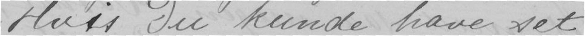Hvis Du kunde have set
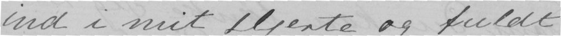ind i mit Hjerte og fuldt
